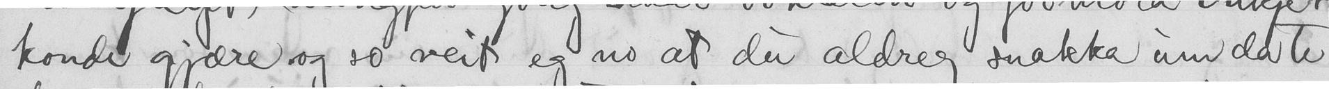konde gjære og so veit eg no at du aldrig snakke um da te
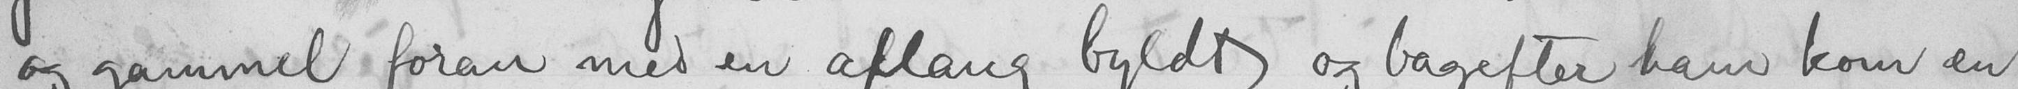og gammel foran med en aflang byldt og bagefter ham kom en
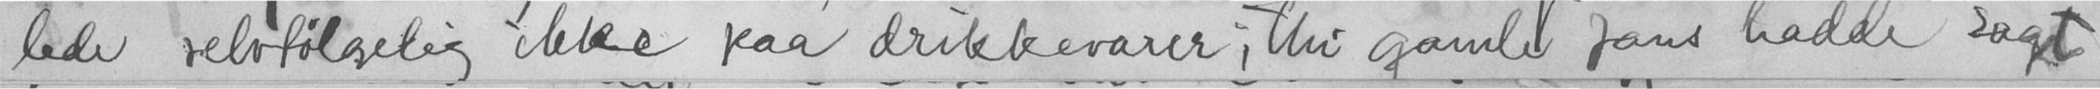lede selvfølgelig ikke paa drikkevarer; thi gamle Jans hadde sagt
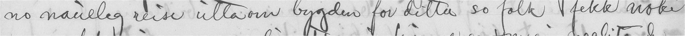no naueleg reise utta om bygden for ditta so folk fekk noke
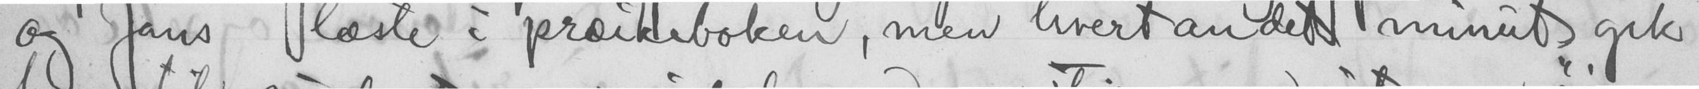og Jans læste i præikeboken, men hvertandet minut gik
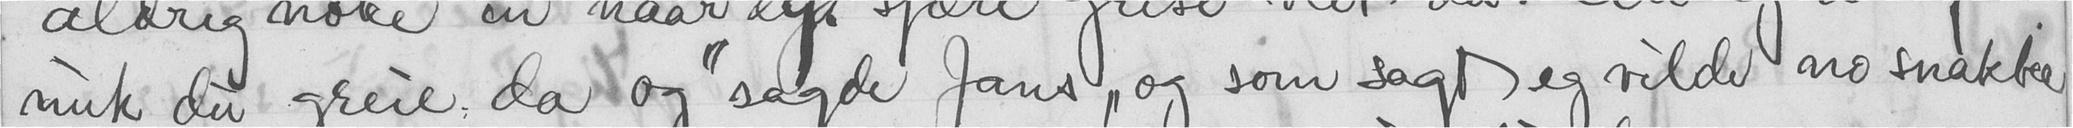nuk du greie da og" sagde Jans "og som sagt eg vilde no snakke
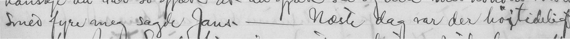smed fyre meg sagde Jans. - Næste dag var der høitideligt
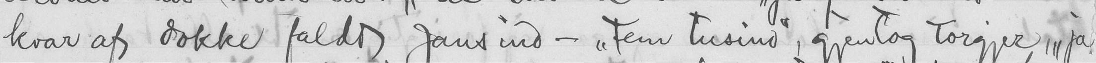kvar af dokke faldt Jans ind - "Fem tusind", gjentog torgjer, "ja
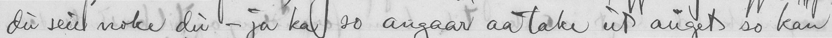du seie noke du - ja ka so angaar aa take ut auget so kan
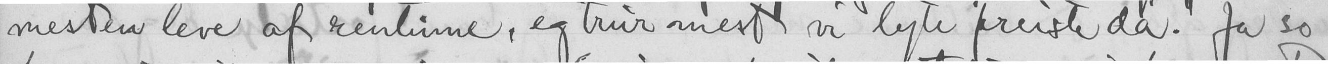mesten leve af rentirne, eg trur mest vi lyte freiste da." Ja so
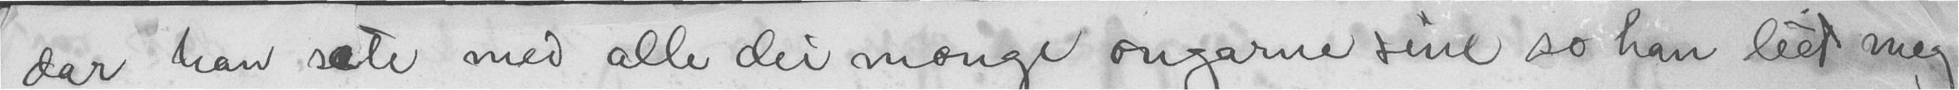dar han sate med alle dei mange ongarne sine so han leet meg
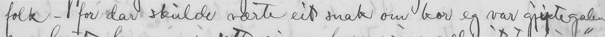folk - for dar skulde værte eit snak om kor eg var gjiptegalen
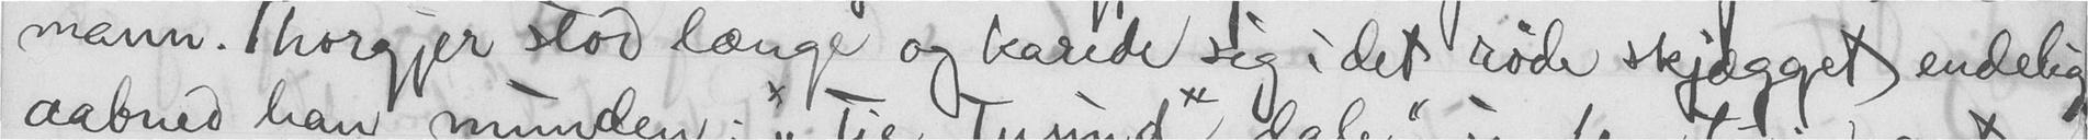mann. Thorgjer stod længe og karede sig, det røde skjægget endelig
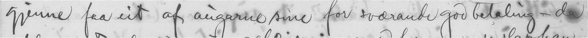gjenne faa eit af augarne sine for sværande god betalnig - da
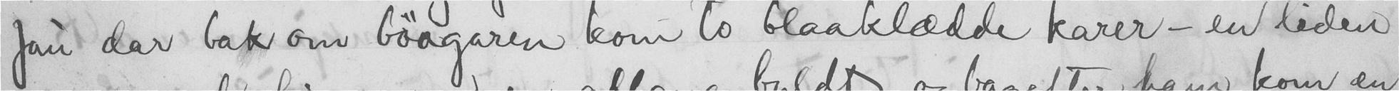Jau dar bak om bøagaren kom to blaaklædde karer - en liden
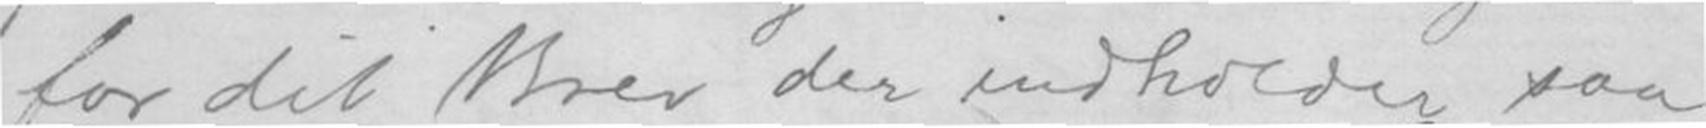for dit Brev der indholder saa
